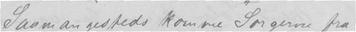Saamangesteds komme Sorgerne fra,
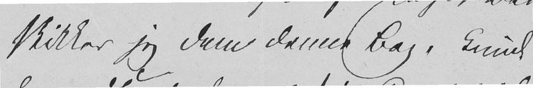skikker jeg dem denne Bog, kunde
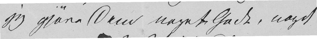jeg gjøre Dem noget Godt, noget
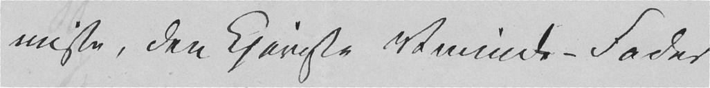miste, den kjereste Veninde – Fader
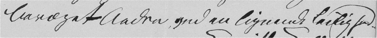bevæget Andre, ved en lignende Leilighed.
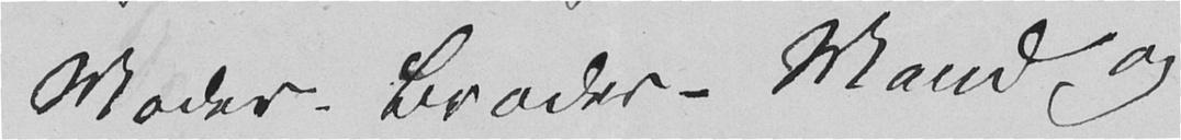Moder – Broder – Mand og
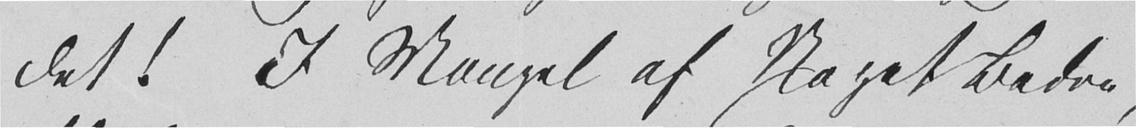det! I Mangel af Noget Bedre
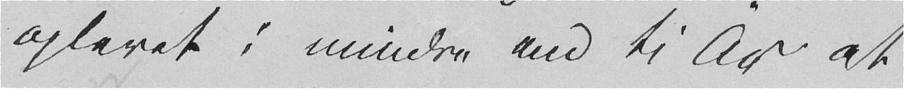oplevet i mindre end ti År at
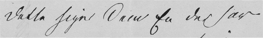Dette siger Dem En der har
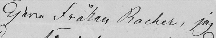Kjere Frøken Backer, jeg
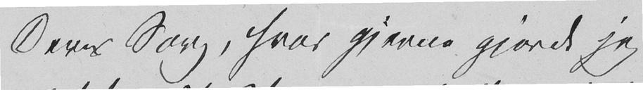Deres Sorg, hvor gjerne gjorde jeg
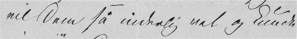vil Dem så inderlig vel og kunde
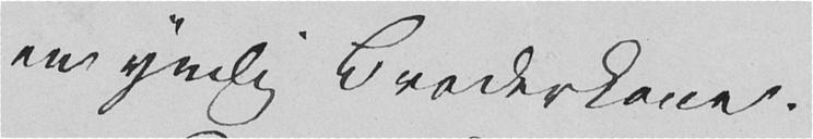en yndig Broderkone.
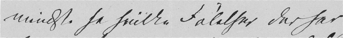mindste se hvilke Følelser der har
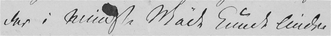der i mindste Måde kunde lindre
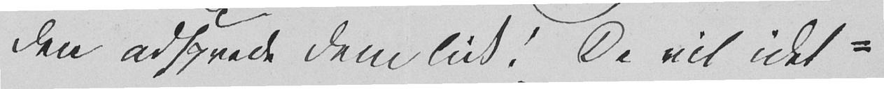den adsprede Dem lidt! De vil idet-
